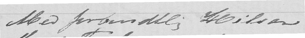Med forbindlig Hilsen
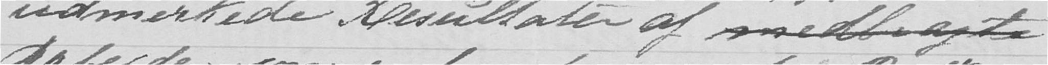udmerkede Resultaler af medbragte
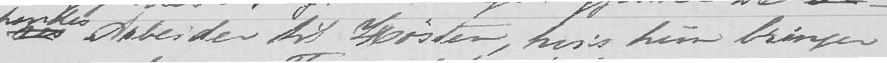res Arbeider til Høsten, hvis hun bringer
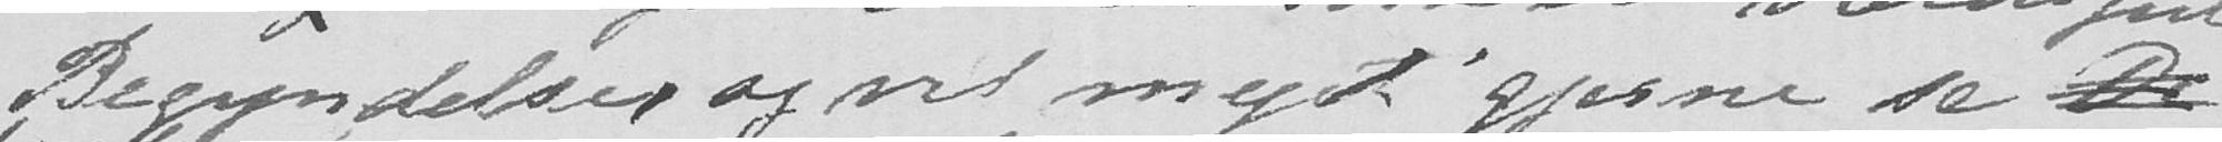Begyndelse, og vil meget gjerne se De-
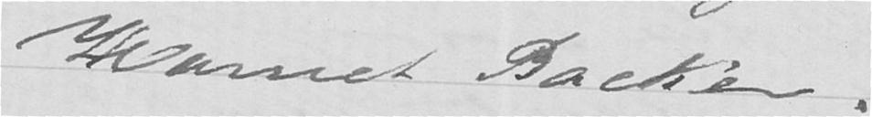Harriet Backer.
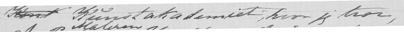Kunst Kunstakademiet, hvor jeg tror,
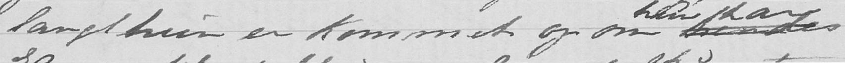langt hun er kommet og om hendes
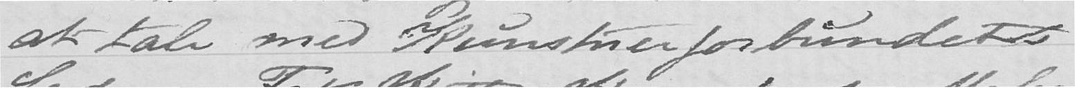at tale med Kunstnerforbundets
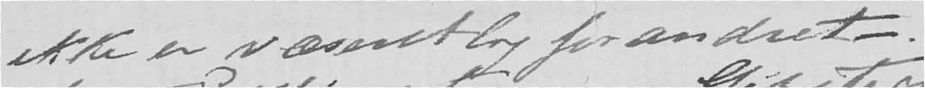ikke er væsentlig forandret -.
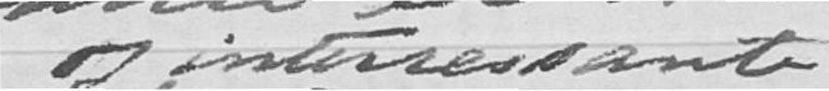og interressante
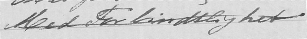Med forbindlighet
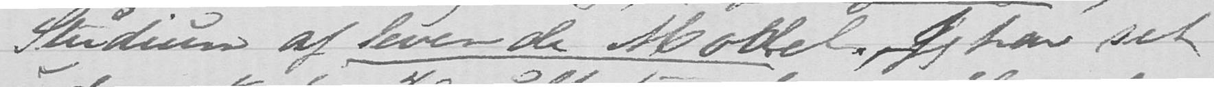Studium af levende Model. Jeg har set
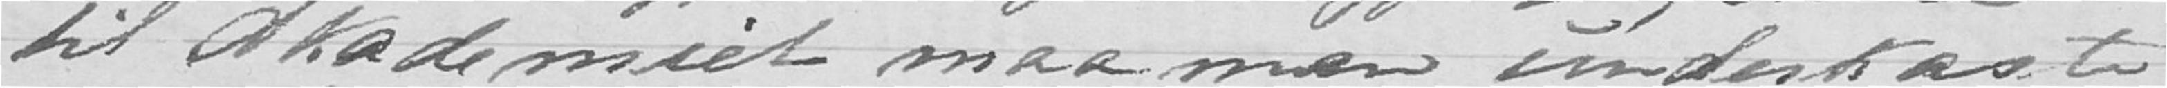til Akademiet maa man underkaste
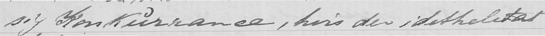sig Konkurrance, hvis der idetheletat
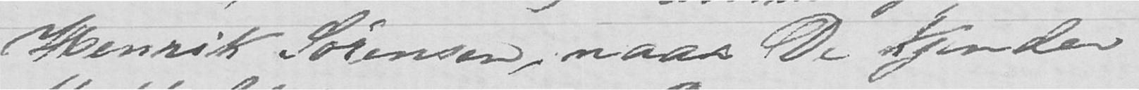Henrik Sørensen, naar De kjender
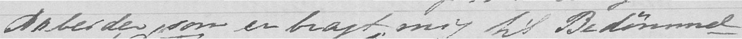Arbeider, som er bragt mig hi Bedømmel
